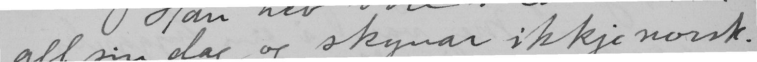all sin dag og skynar ikkje norsk.
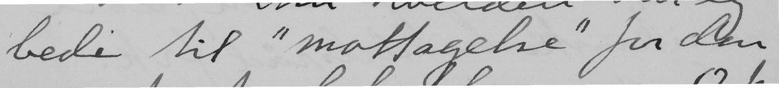bedi til "mottagelse" for den
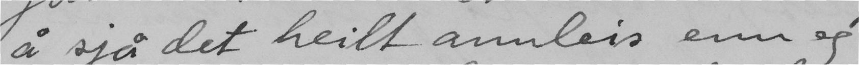å sjå det heilt annleis enn eg
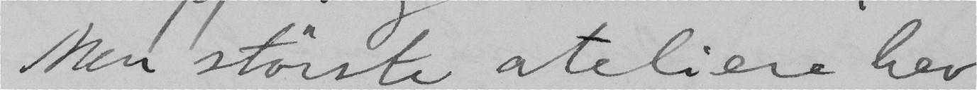Men störste ateliere hev
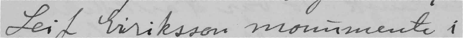Leif Eiriksson monumente i
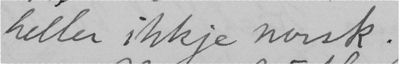heller ikkje norsk.
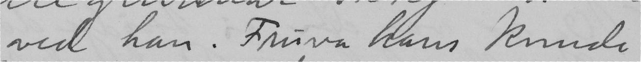i med han. Fruva hans kunde
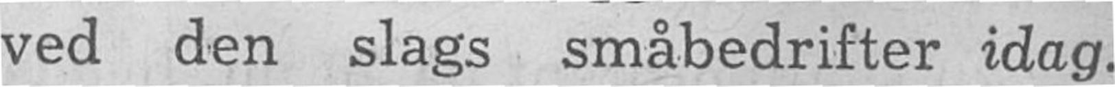ved den slags småbedrifter idag.
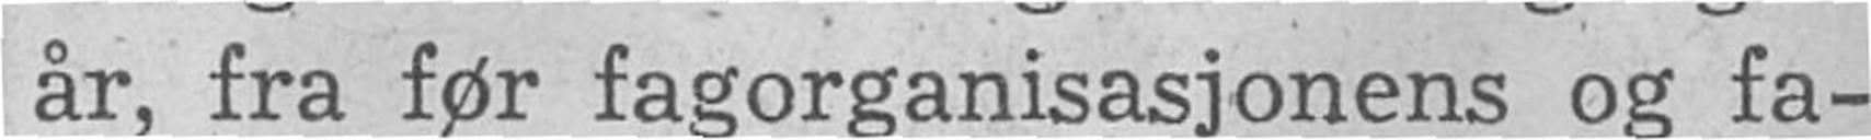år, fra før fagorganisasjonens og fa-
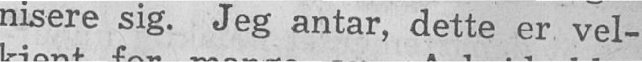nisere sig. Jeg antar, dette er vel-
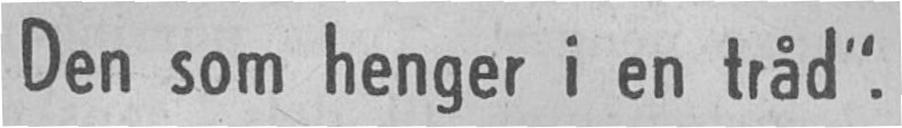"Den som henger i en tråd“
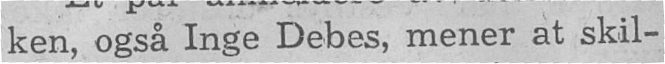ken, også Inge Debes, mener at skil-
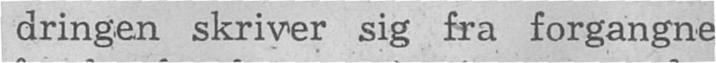dringen skriver sig fra forgangne
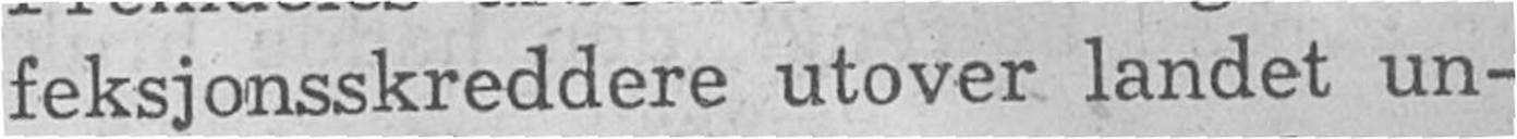feksjonsskreddere utover landet un-
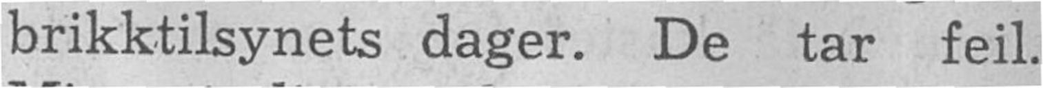brikktilsynets dager. De tar feil.
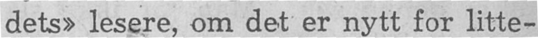dets" lesere, om det er nytt for litte-
